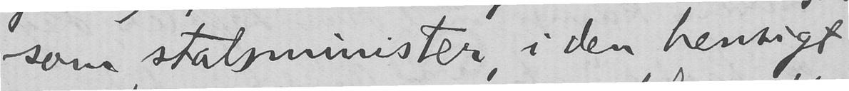som statsminister, i den hensigt
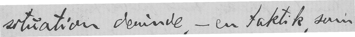situation derinde, - en taktik, som
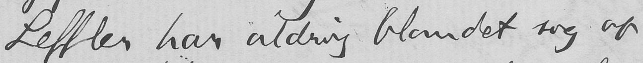Leffler har aldrig blandet sig op
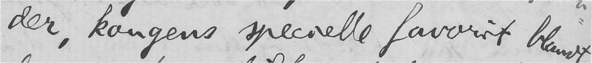der, kongens specielle favorit blandt
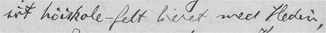sit høiskole-felt lieret med Hedin,
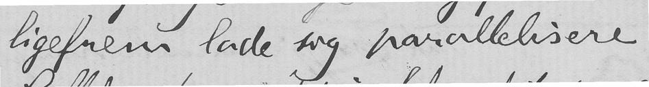ligefrem lade sig parallelisere.
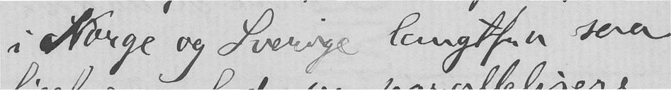i Norge og Sverige langtfra saa
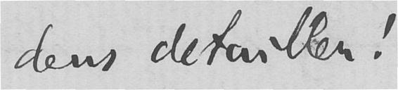dens detailler!
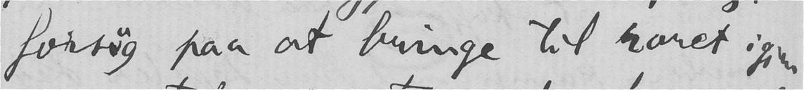forsøg paa at bringe til roret igjen
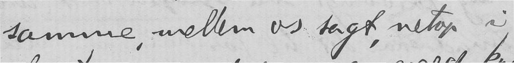samme, mellem os sagt, netop i
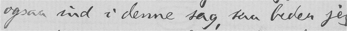ogsaa ind i denne sag, saa beder jeg
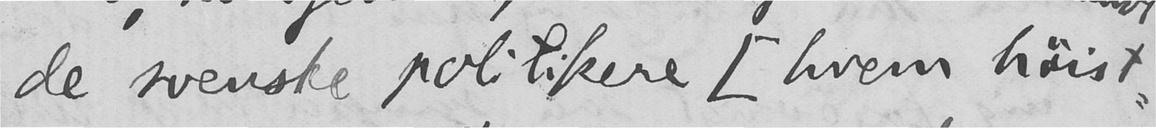de svenske politikere [hvem høist-
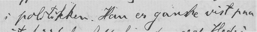i politikken. Han er ganske vist paa
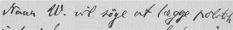Naar W. vil søge at lægge politik
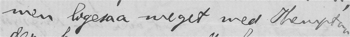men ligesaa meget med Themptan-
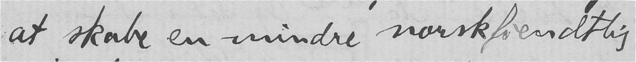at skabe en mindre norskfiendtlig
